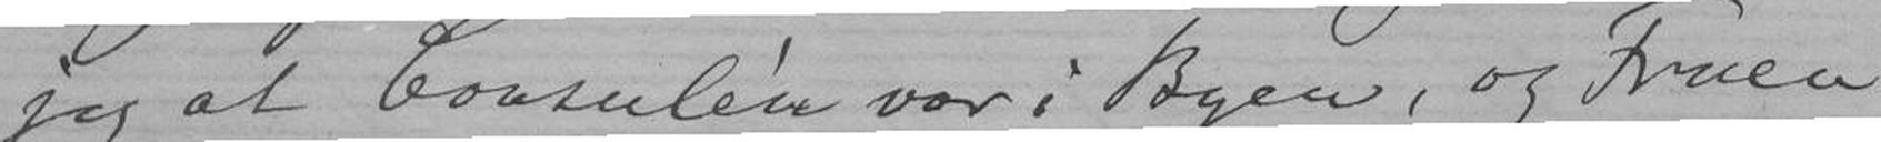jeg at Consulèn var i Byen, og Fruen
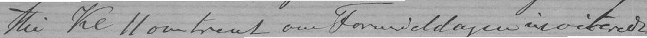Thi Kl 11 omtrent om Formiddagen inviterede
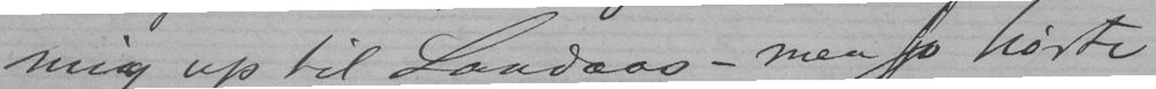mig up til Landaas - men so hørte
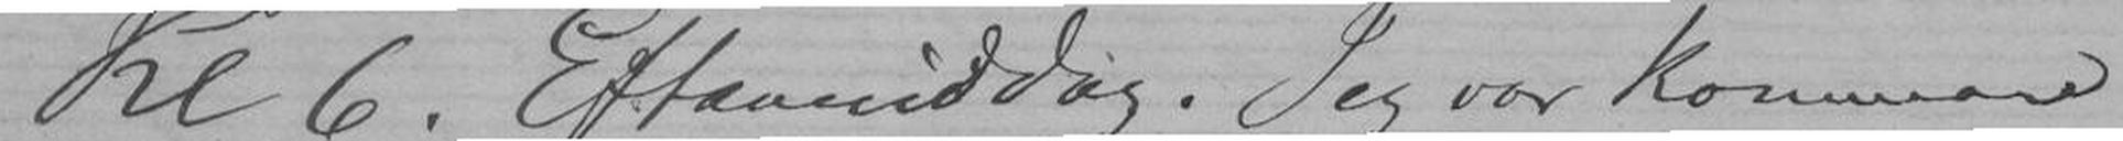Kl 6. Eftermiddag. Jeg var kommed
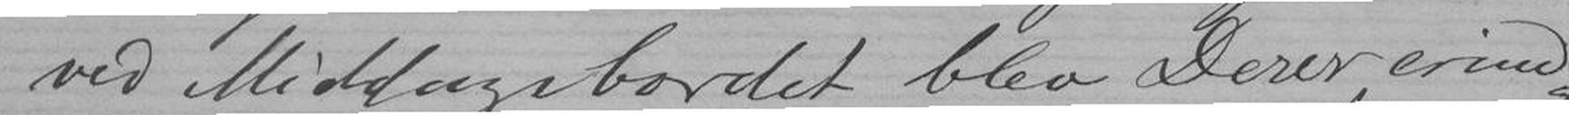ved Middagsbordet blev Deres erind
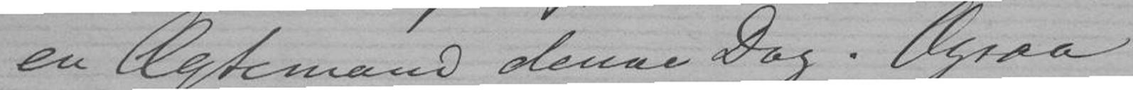en Ægtemand denne Dag. Ogsaa
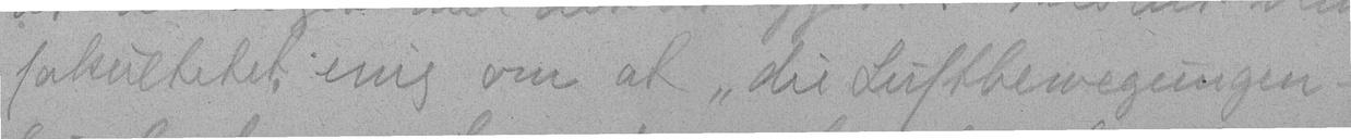fakultetet enig om at "die Luftbewegungen- -"
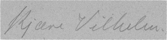Kjære Vilhelm!
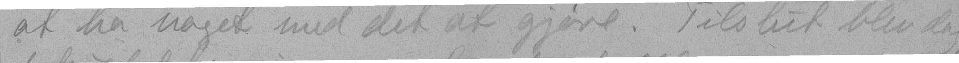at ha noget med det at gjøre. Tilslut blev dog
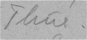Thue.
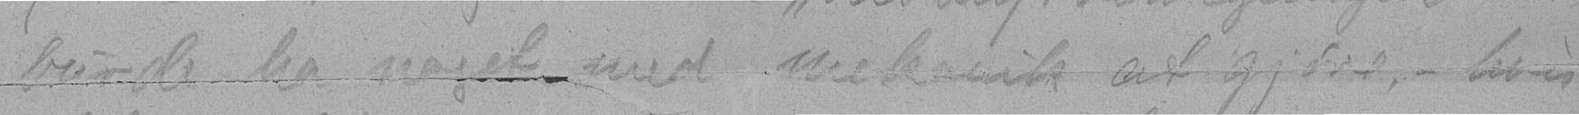burde ha noget med mekanik at gjøre, - hvis
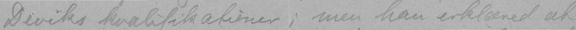Deviks kvalifikationer; men han erklæred at
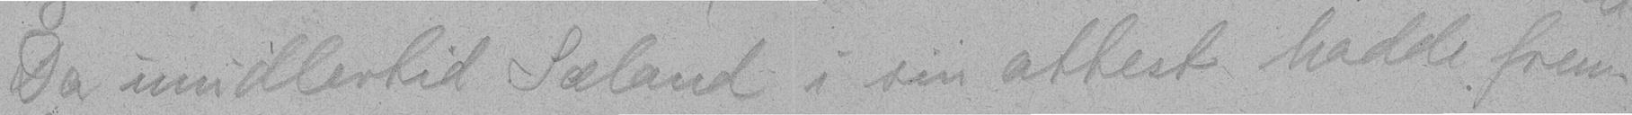Da imidlertid Sæland i sin attest hadde frem-
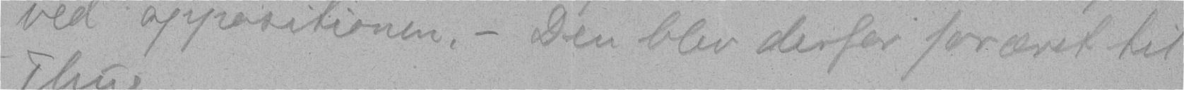ved oppositionen, - Den blev derfor foræret til
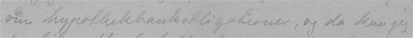om hypothekbankobligationer, og da kan jeg
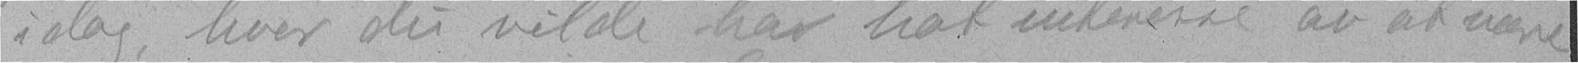i dag, hvor du vilde har hat interesse av at være
